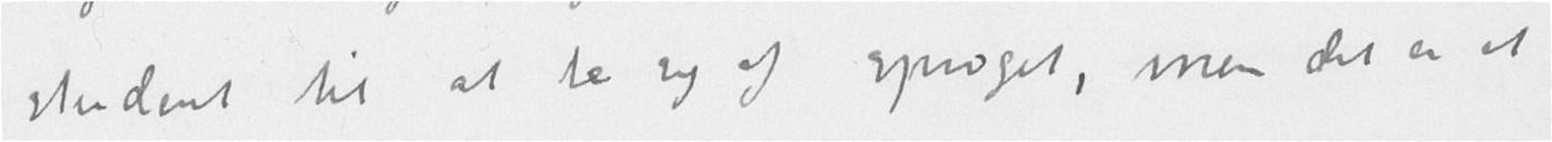student til at ta sig af sproget, men det er et
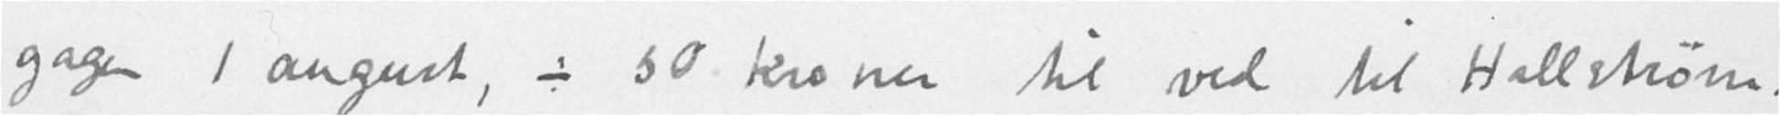gagen 1 august, ÷ 50 kroner til ved til Hallstrøm.
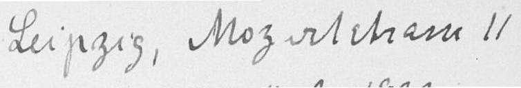Leipzig, Mozartstrasse II
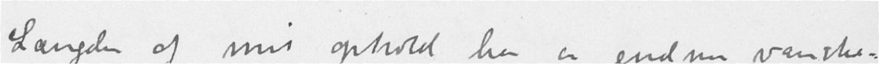Længden af mit ophold her er endnu vanske-
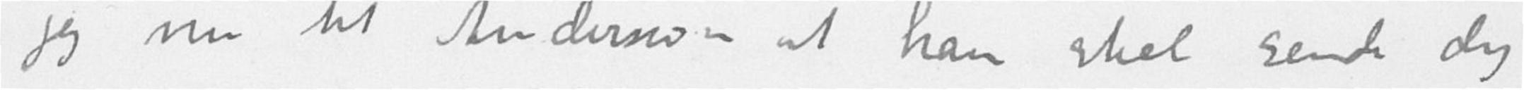jeg nu til Andersson at han skal sende dig
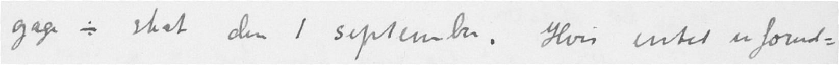gage ÷ skat den 1 september. Hvis intet uforud-
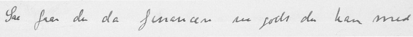Saa faar du da financere saa godt du kan med
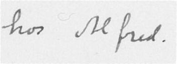hos Alfred.
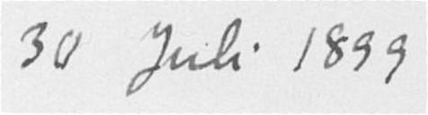30 Juli 1899
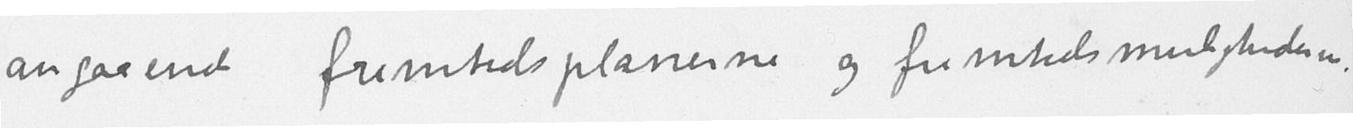angaaende fremtidsplanerne og fremtidsmulighederne.
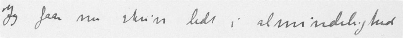Jeg faar nu skrive lidt i almindelighed
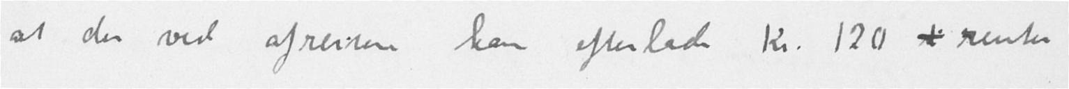at du ved afreisen kan efterlade Kr. 120 + renter
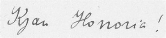Kjære Honoria!
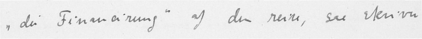"die Financirung" af din reise, saa skriver
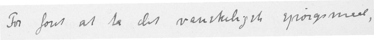For først at ta det vanskeligste spørgsmaal,
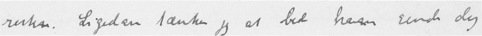resten. Ligedan tænker jeg at bede ham sende dig
